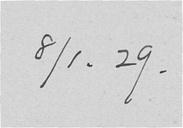8/1. 29.
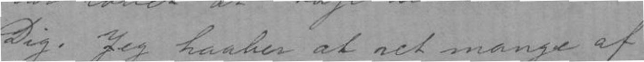Dig. Jeg haaber at ret mange af
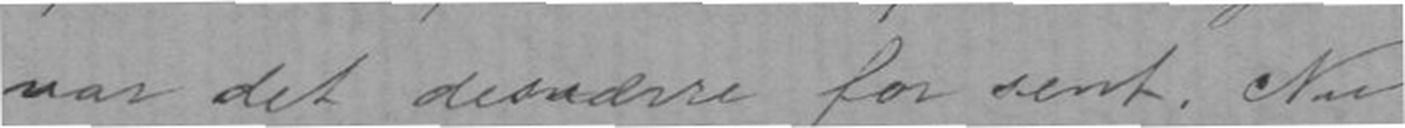var det desværre for sent. Nu
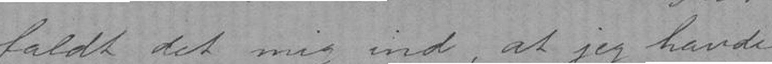faldt det mig ind, at jeg havde
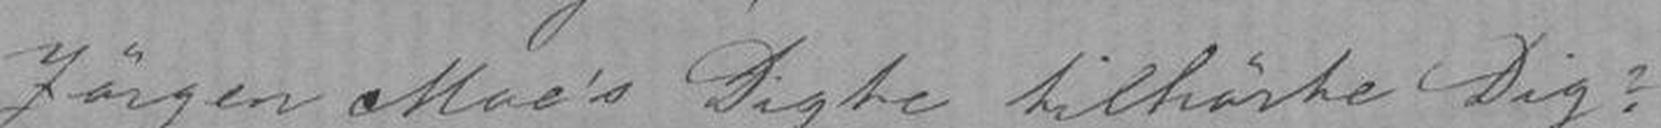Jørgen Moe's Digte tilhørte Dig?
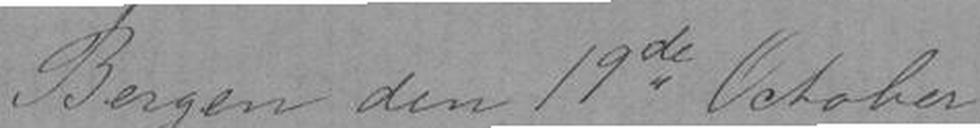Bergen den 19de October
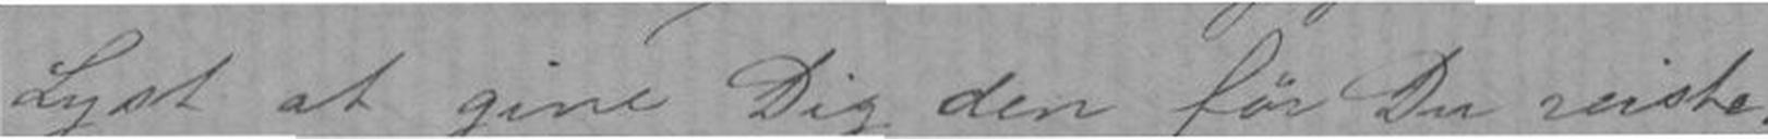Lyst at give dig den før Du reiste;
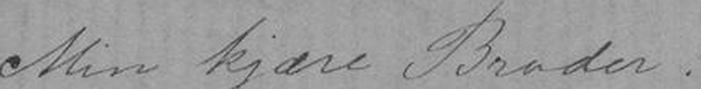Min kjære Broder!
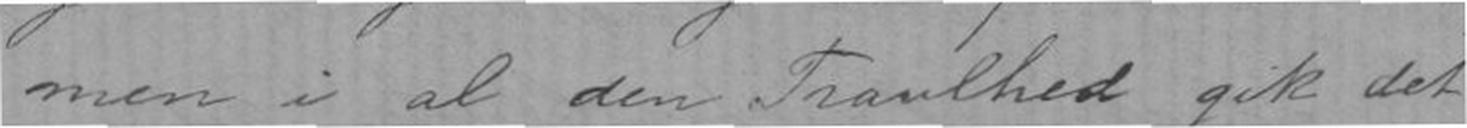men i al den Travlhed gik det
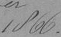1866
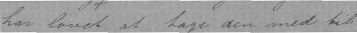har lovet at tage den med til
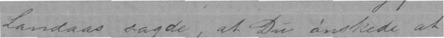Landaas sagde, at Du ønskede at
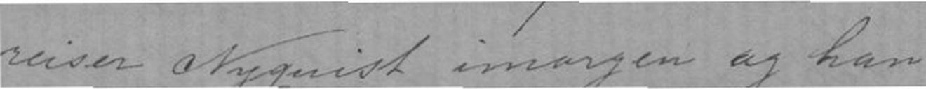reiser Nyquist imorgen og han
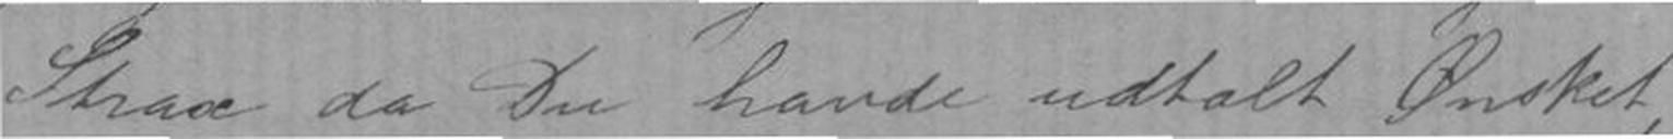Strax da Du havde udtalt Ønsket,
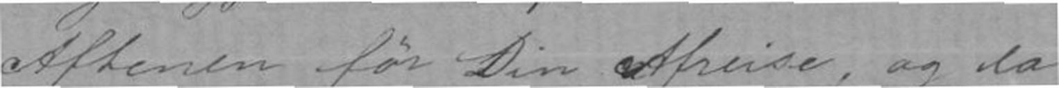Aftenen før Din Afreise, og da
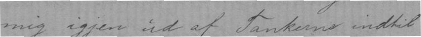mig igjen ud af Tankerne indtil
